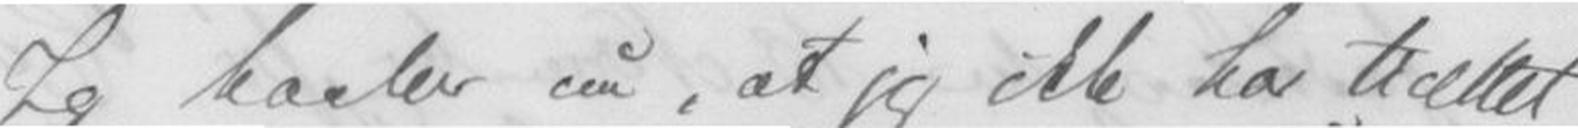Jeg haaber nu, at jeg ikke har trættet
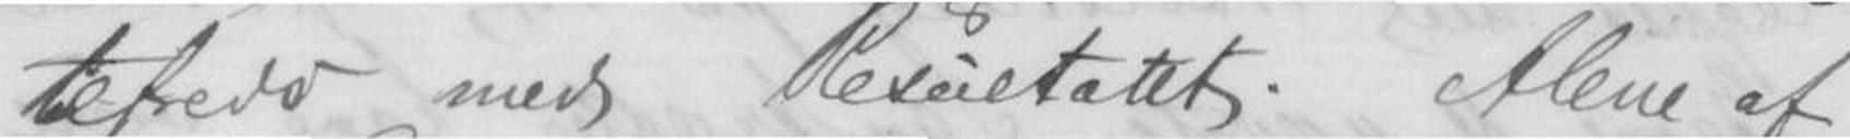tilfreds med Resultatet. Alene af
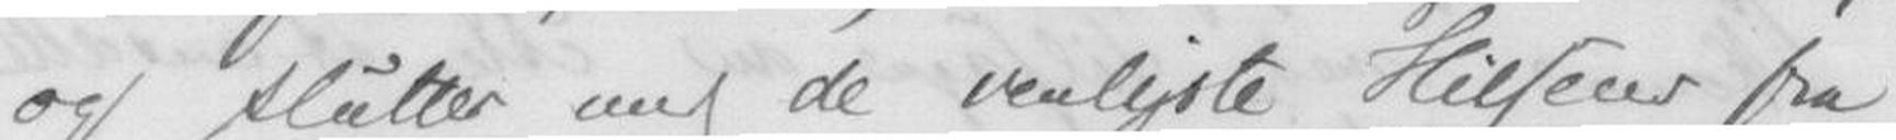og slutter med de venligste Hilsener fra
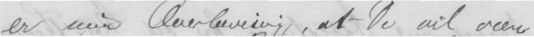er min Overbevisning, at De vil være
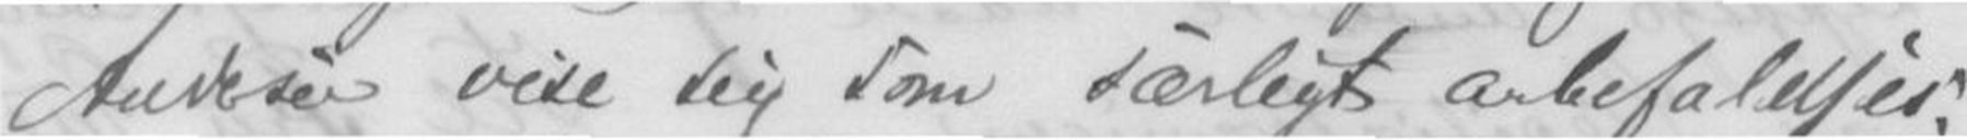Andersen veie sig som særlit anbefalings-
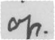op.
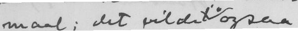maal; det vilde jo ogsaa
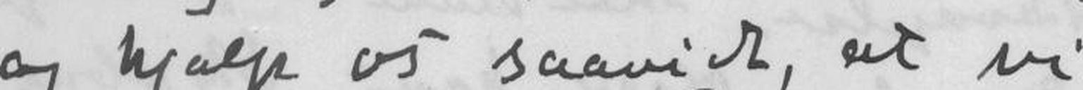og hjalp os saavidt, at vi
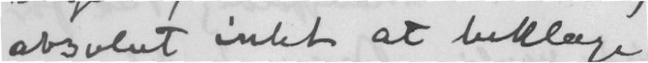absolut intet at beklage
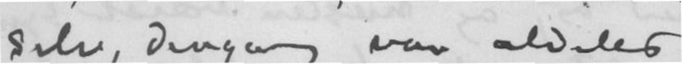selv, dengang var aldeles
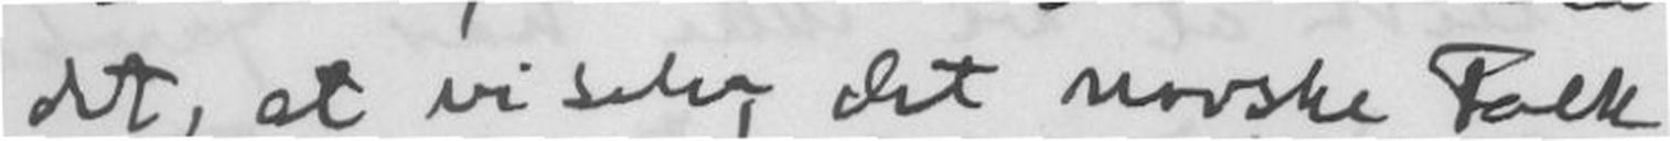det, at vi selv, det norske Folk
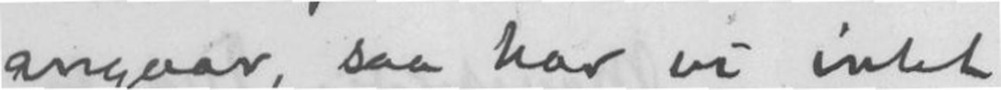angaar, saa har vi intet,
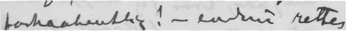- forhaabentlig! - endnu rettes
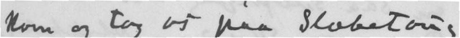kom og tog os paa Slæbetoug
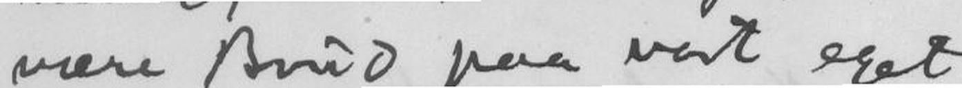være Brud paa vort eget
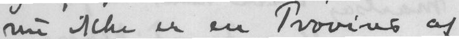nu ikke er en Provins af
